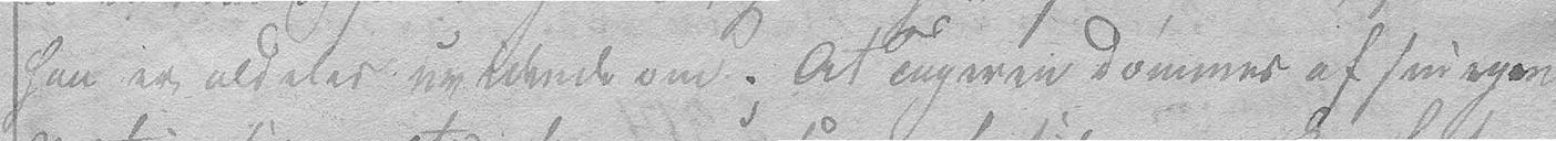han er aldeles uvidende om. At Tugeren dømmes af sin egen
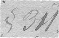§ 311.
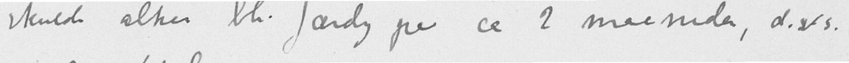skulde altsaa bli færdig paa ca 2 maaneder, d.v.s.
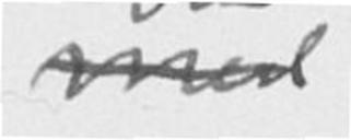med
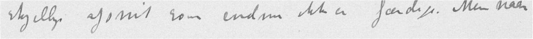skjellige afsnit som endnu ikke er færdige. Men naar
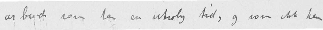arbeide som tar en utrolig tid, og som ikke kan
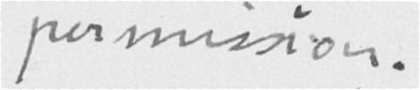permission.
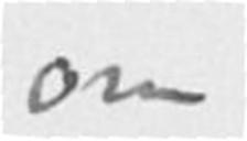om
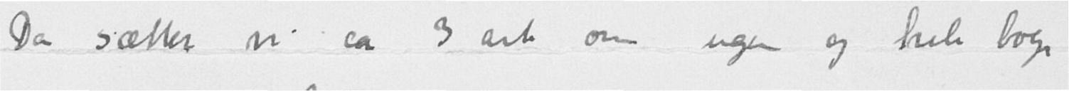Da sætter vi ca 3 ark om ugen og hele bogen
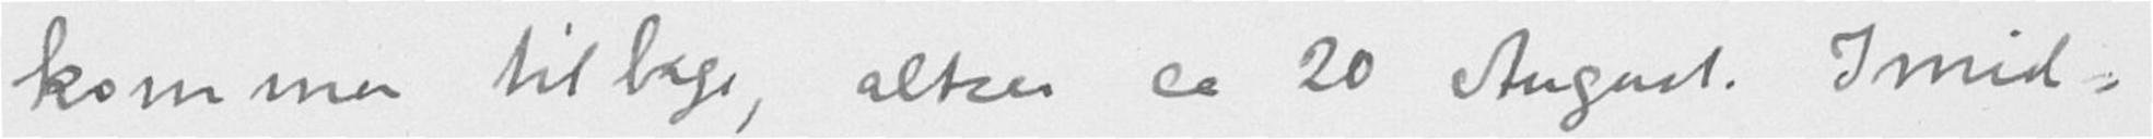kommer tilbage, altsaa ca 20 August. Imid-
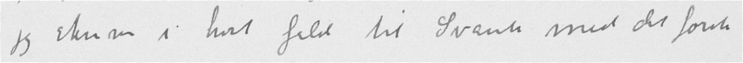jeg skriver i hvert fald til Svante med det første
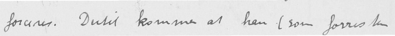forceres. Dertil kommer at han (som forresten
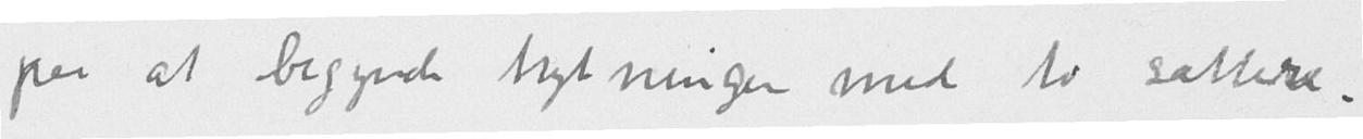paa at begynde trykningen med to sættere.
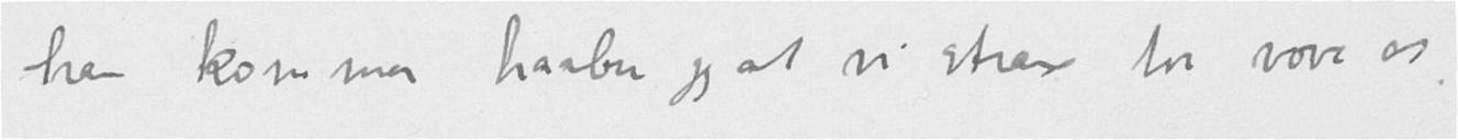han kommer haaber jeg at vi strax tør vove os
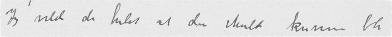Jeg vilde da helst at du skulde kunne bli
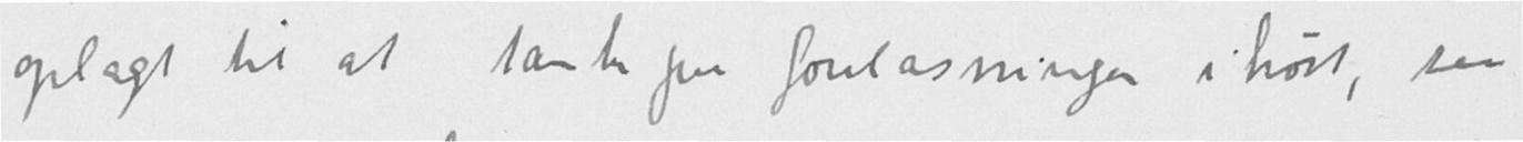oplagt til at tænke paa forelæsninger i høst, saa
